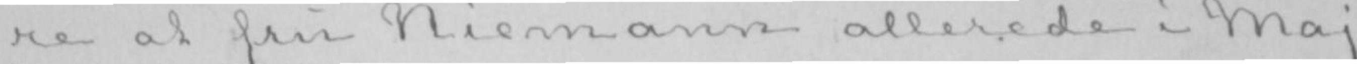re at fru Niemann allerede i Maj
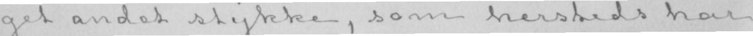get andet stykke, som hersteds har
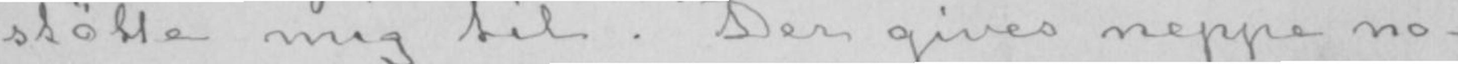støtte mig til. Det gives neppe no-
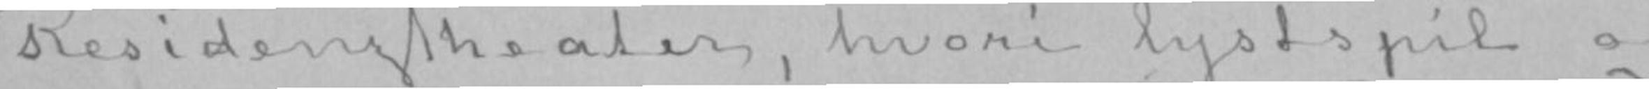Residenztheater, hvori lystspil og
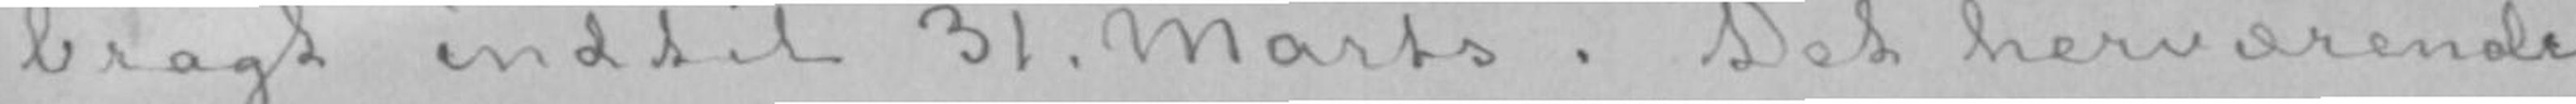bragt indtil 31. Marts. Det herværende
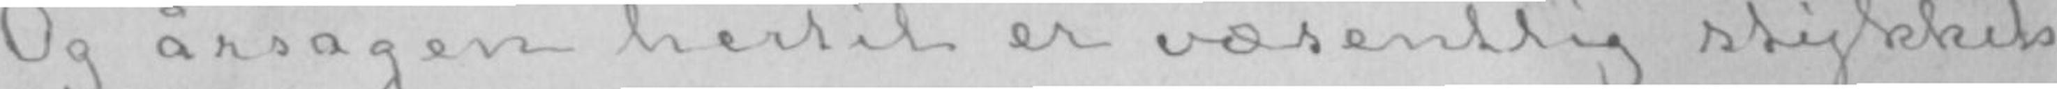Og årsagen hertil er væsentlig stykkets
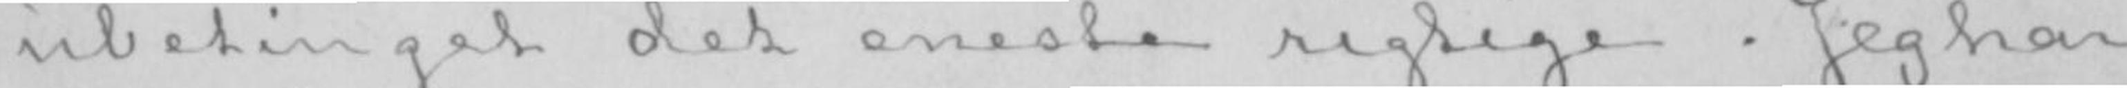ubetinget det eneste rigtige. Jeg har
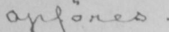opføres.
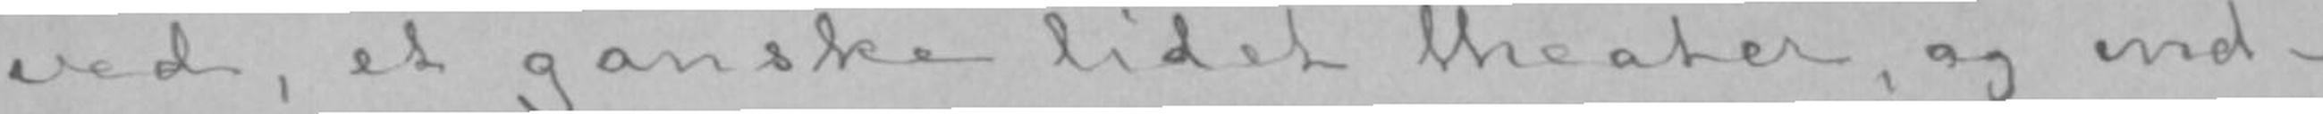ved, et ganske lidet theater, og ind-
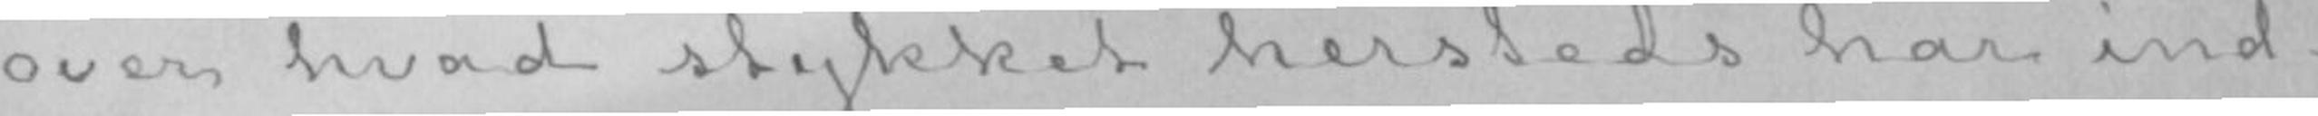over hvad stykket hersteds har ind-
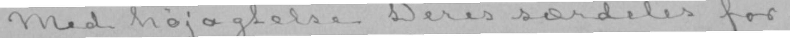Med højagtelse Deres særdeles for-
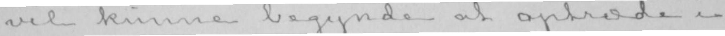vil kunne begynde at optræde i
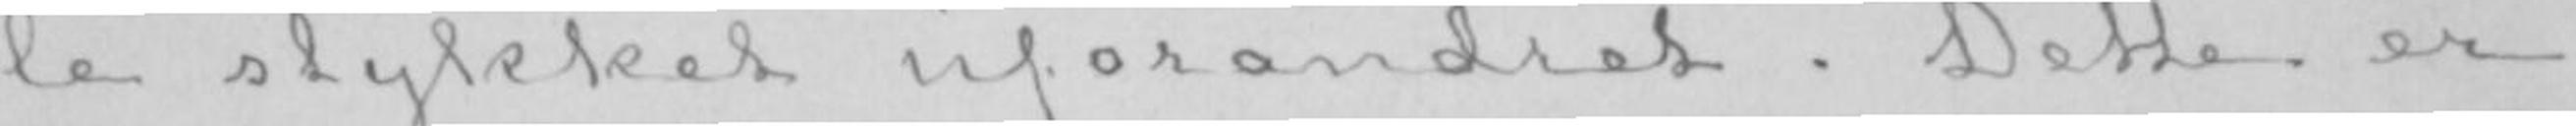le stykket uforandret. Dette er
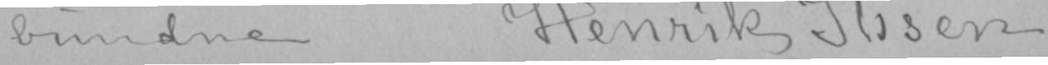bundne Henrik Ibsen.
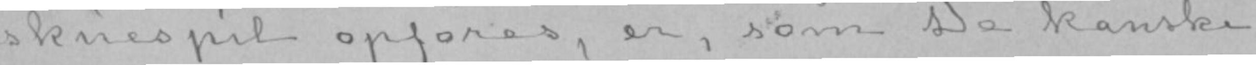skuespil opføres, er, som De kanske
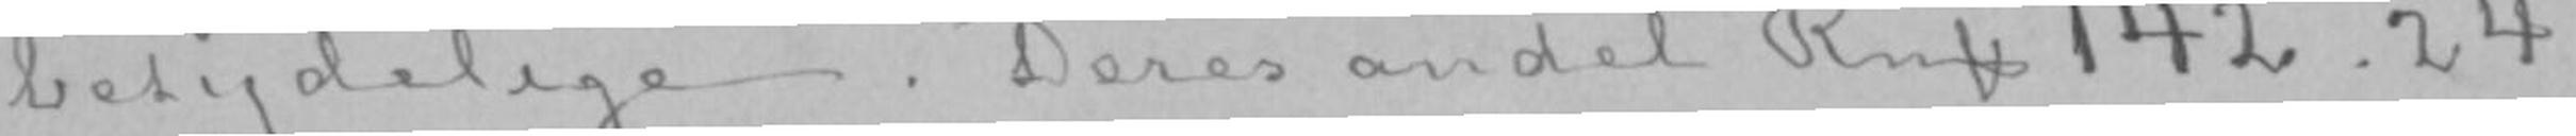betydelige. Deres andel RmՖ 142.24
